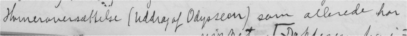Homeroversættelse (Uddrag af Odysseen) som allerede har
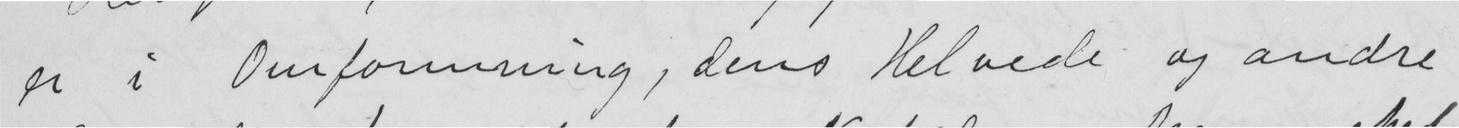er i Omformning, dens Helvede og andre
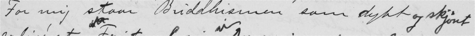For mig staar Buddhismen som dybt og skjønt
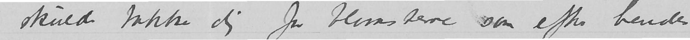Jeg skulde takke dig for blomsterne som efter hendes
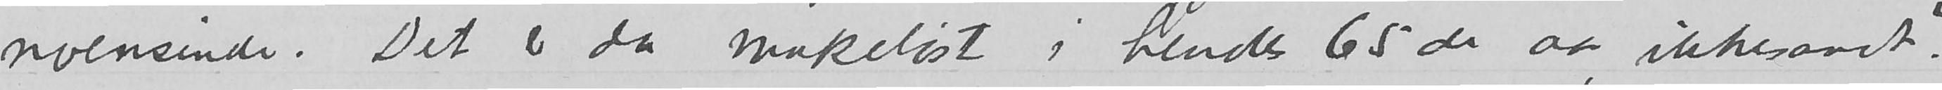noensinde. Det er da makeløst i hendes 65de aar, ikkesandt?
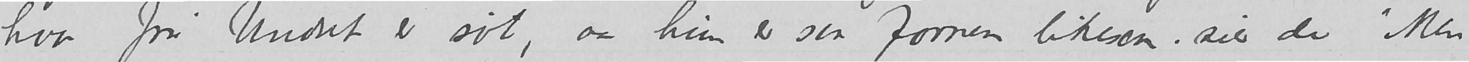hvor fru Undset er søt, aa hun er saa fornem likesom, sier de«Men
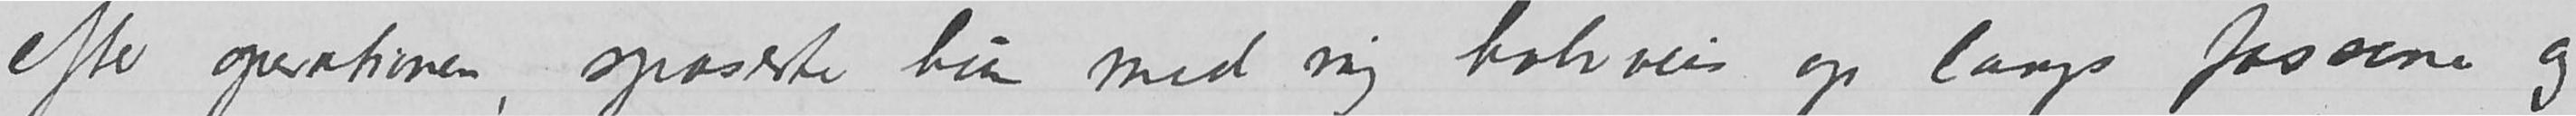efter operationen, spaserte hun med mig halvveis op langs fossene og
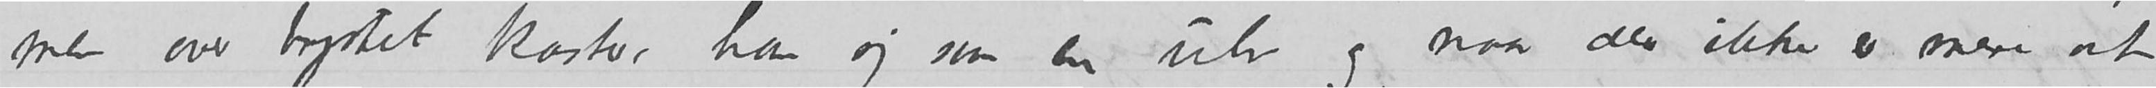men over brystet kaster han sig som en ulv og naar dert ikke er mere at
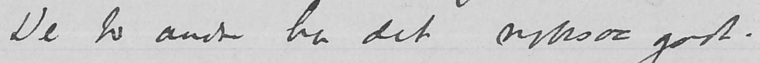De to andre har det noksaa godt.
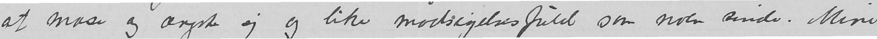at mase og ængste sig og like modsigelsesfuld som noen sinde
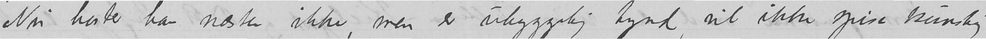Nu hoster han næsten ikke, men er uhyggelig tynd, vil ikke spise kunstig
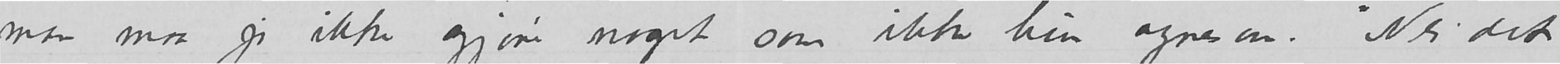man maa jo ikke gjøre noget som ikke hun synes om. «Nei det
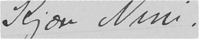Kjære Nini,
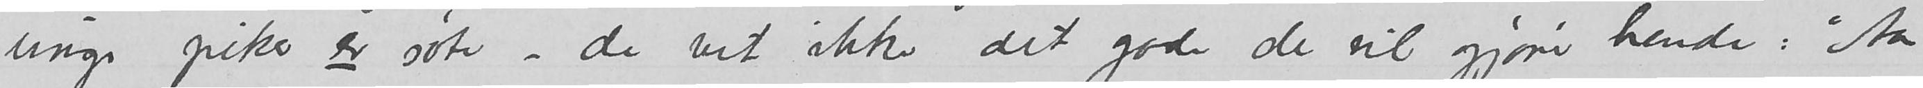Mine unge piker er søte – de vet ikke det gode de vil gjøre hende: «Aa
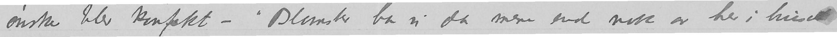ønske blev konfekt – «Blomster har vi da mere end nok av her i huset
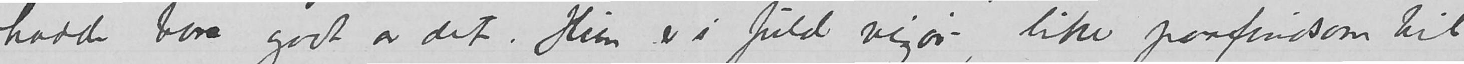hadde bare godt av det.  Hun er i fuld vigør –-, like paafindsom til
